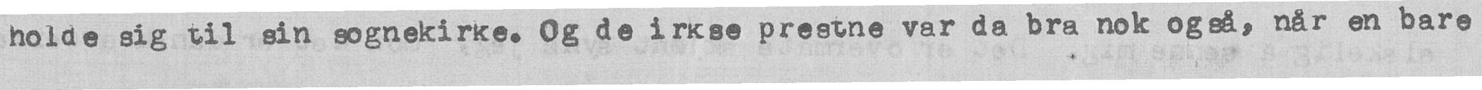holde sig til sin sognekirke. Og de irkse prestne var da bra nok også, når en bare
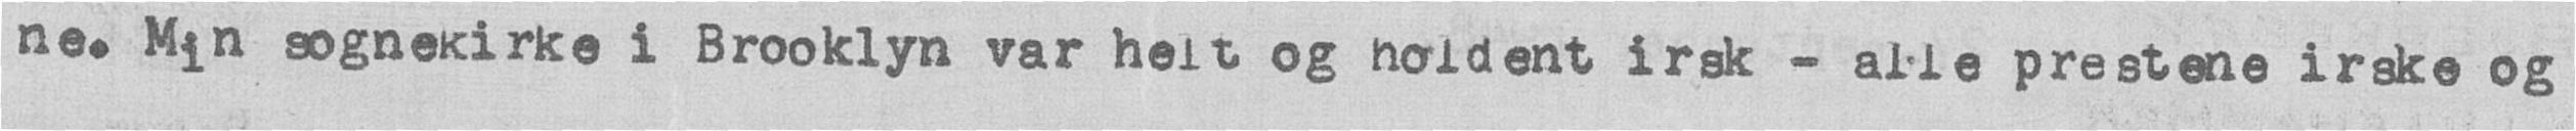ne. Min sognekirke i Brooklyn var helt og holdent irsk - alle prestene irske og
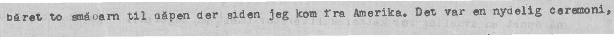båret to småparn til dåpen der siden jeg kom fra Amerika. Det var en nydelig ceremoni,
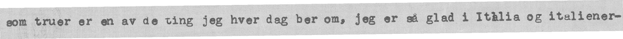som truer er en av de ting jeg hver dag ber om, jeg er så glad i Ithlia og italiener-
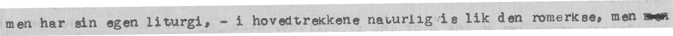men har sin egen liturgi, - i hovedtrekkene naturligwis lik den romerkse, men men
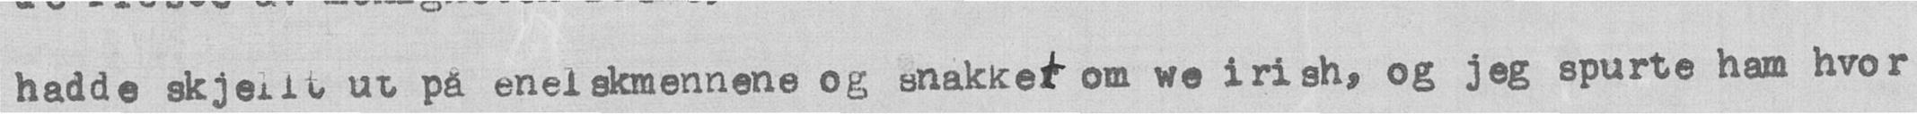hadde skjellt ut på enelskmennene og snakkef om we irish, og jeg spurte ham hvor
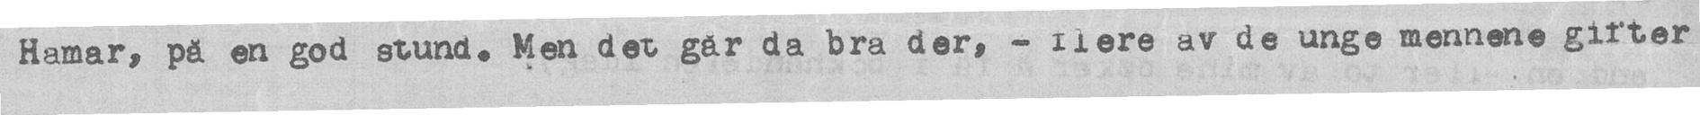Hamar, på en god stund. Men det går da bra der, — flere av de unge mennene gifter
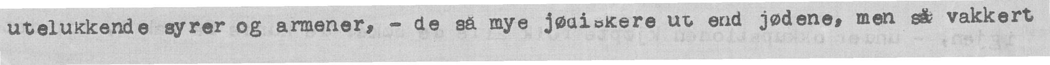utelukkende syrer og armener, - de så mye jødiskere ut end jødene, men s vakkert
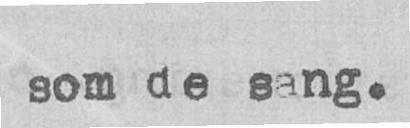som de sang.
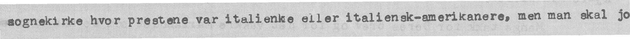sognekirke hvor prestene var italienke eller italiensk-amerikanere, men man skal jo
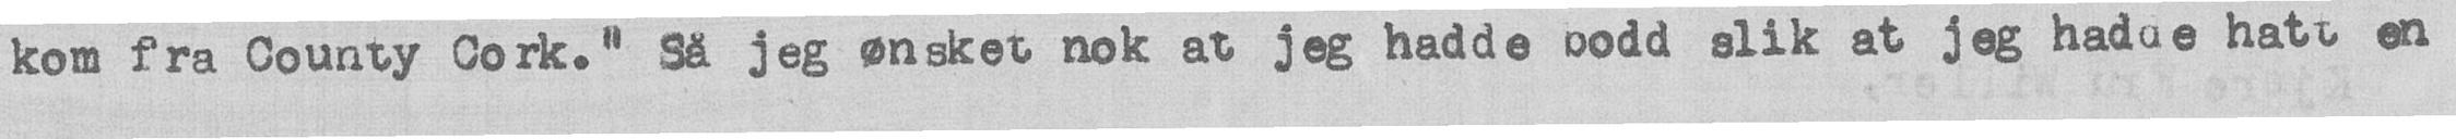kom fra County Cork." Så jeg ønsket nok at jeg hadde bodd slik at jeg hadde hatt en
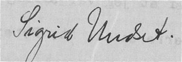Sigrid Undset.
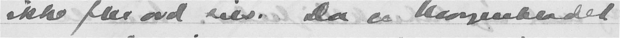ikke fler ord sies. Da er Morgenbladet
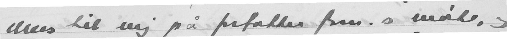ellers til mig på forfatter forn.s møte, og
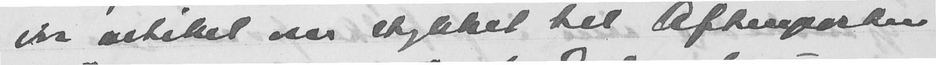en artikel om stykket til Aftenposten
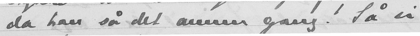da han så det annen gang! Så vi
